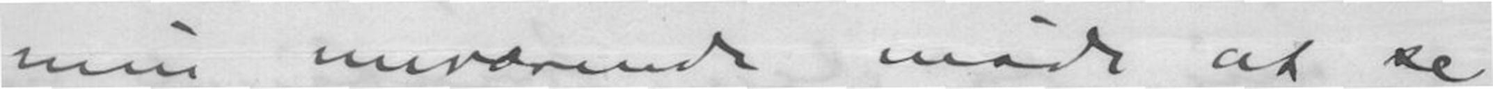min nuværende måde at se
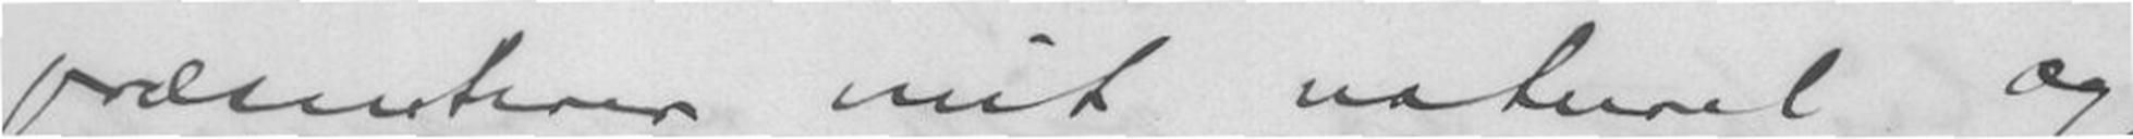præsenterer mit naturel og
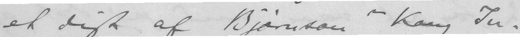et digt af Bjørnson "Kong In-
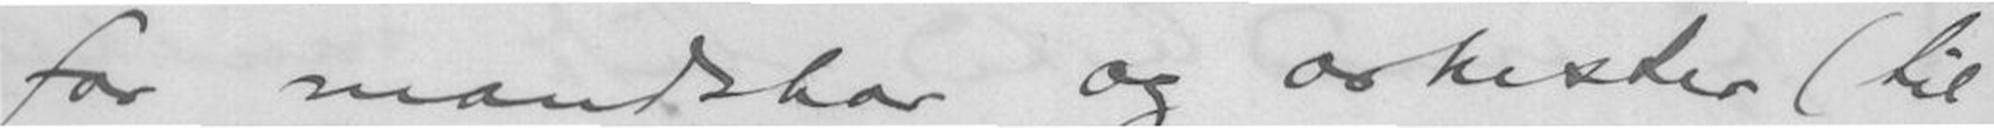for mandskor og orkester (til
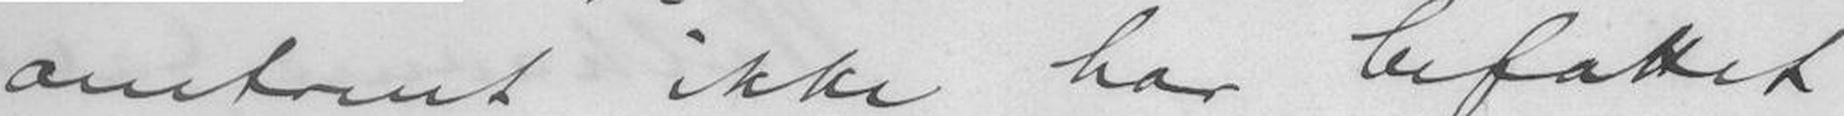omtrent ikke har befattet
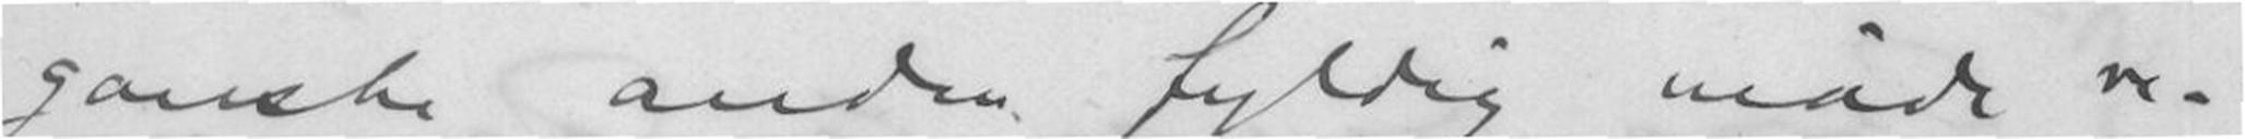ganske anden fyldig måde re-
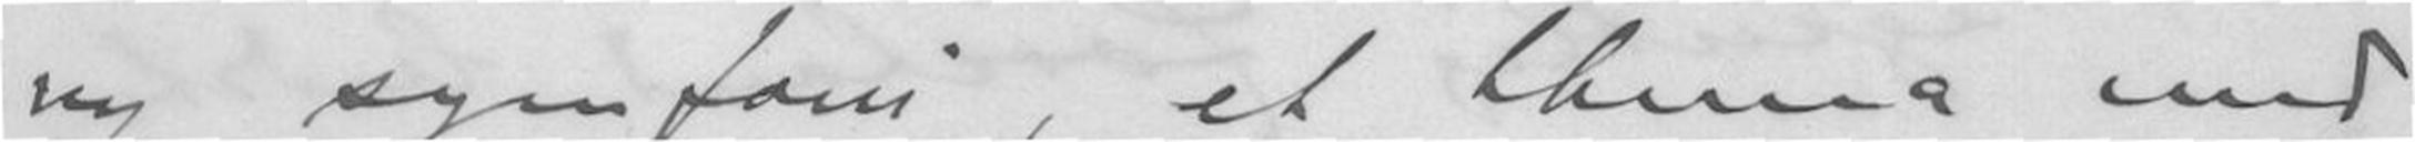ny symfoni, et thema med
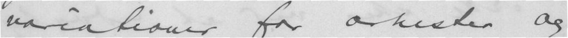variationer for orkester og
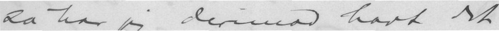så har jeg derimod havt det
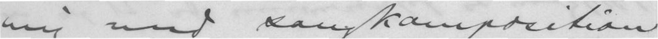mig med sangkompsition,
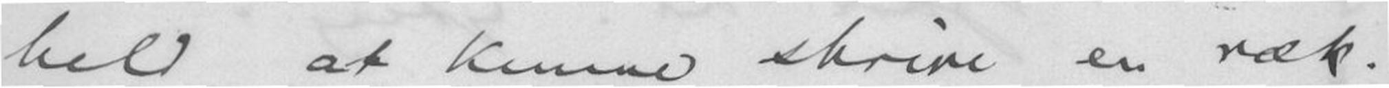held at kunne skrive en ræk-
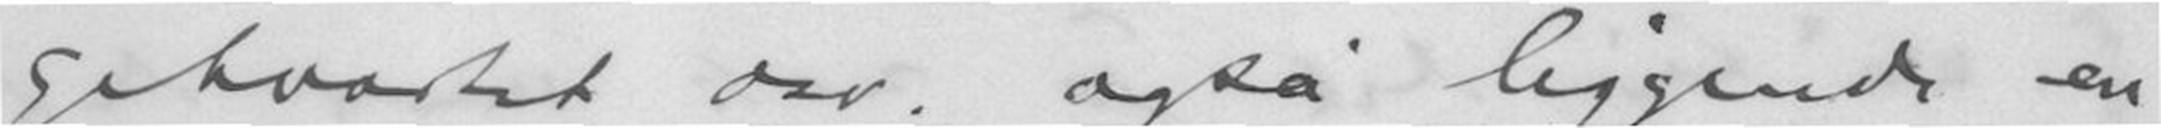gekvartet osv. også liggende en
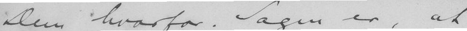Dem hvorfor. Sagen er, at
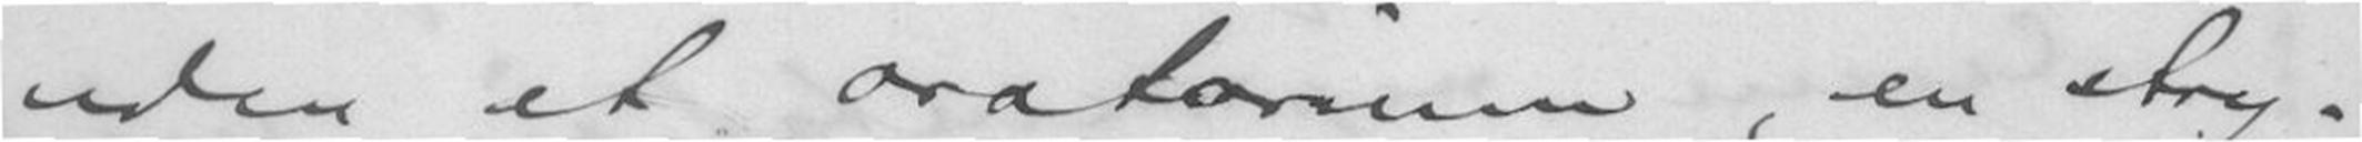uden et oratorium, en stry-
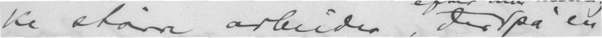ke større arbeider, der på en
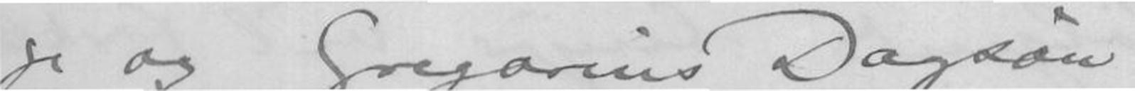ge og Gregorius Dagsøn
